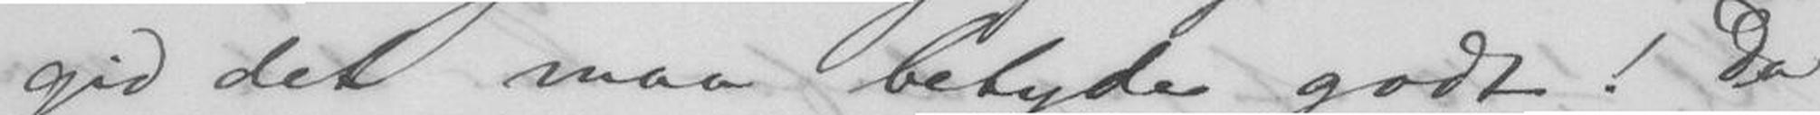gid det maa betyde godt! Da
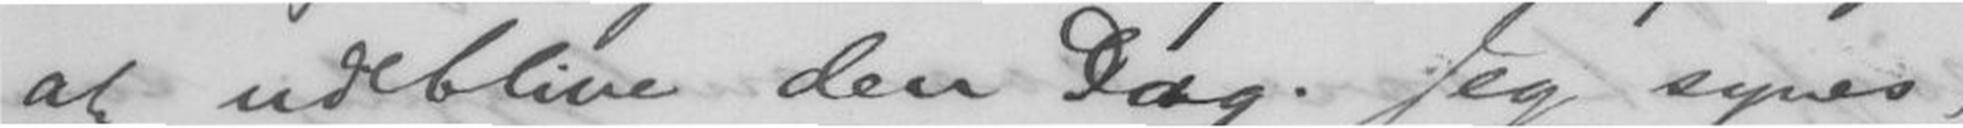at udblive den Dag. Jeg synes,
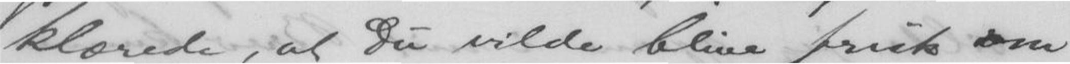klærede, at Du vilde blive frisk om
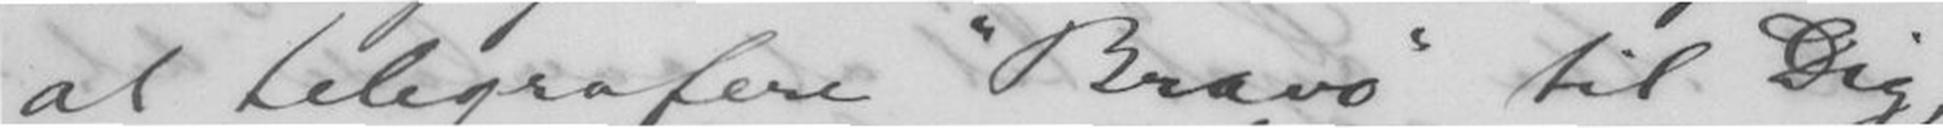at telegrafere "Bravo" til Dig,
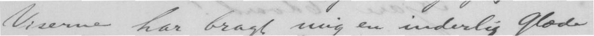Viserne har bragt mig en inderlig Glæde
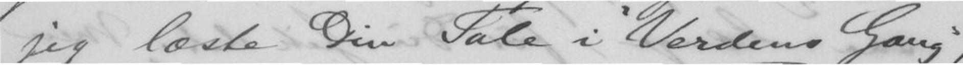jeg læste Din Tale i "Verdens Gang",
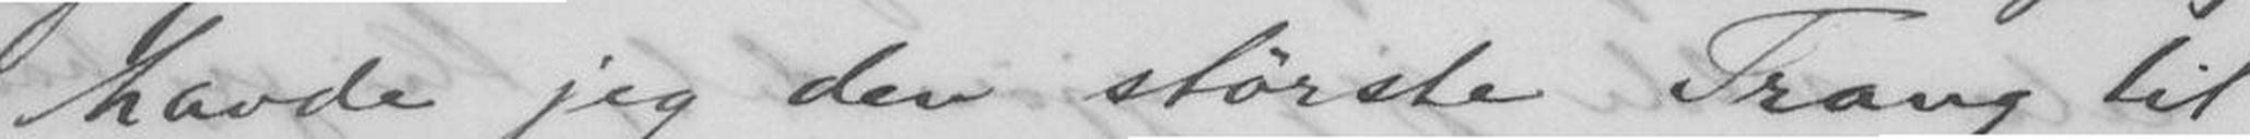havde jeg den største Trang til
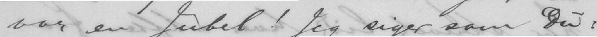var en Jubel! Jeg siger som Du:
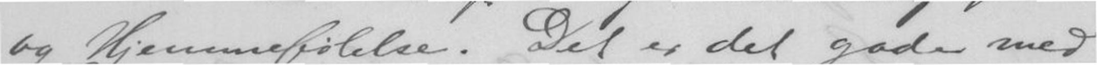og Hjemmefølelse. Det er det gode med
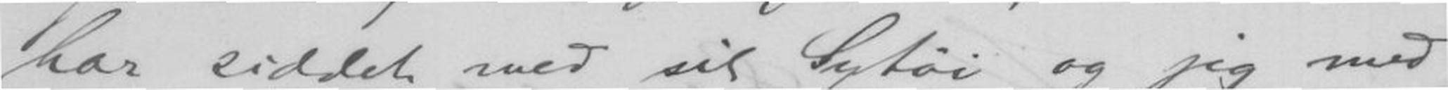har siddet med sit Sytøi og jeg med
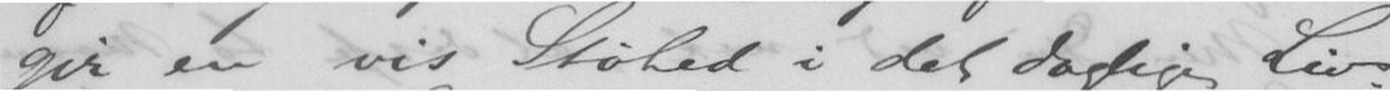gir en vis Støhed i det daglige Liv.
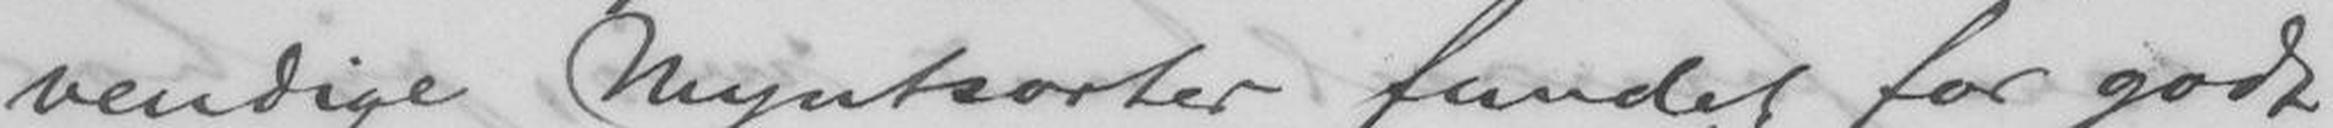vendige Myntsorter fundet for godt
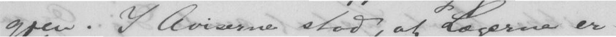gjen. I Aviserne stod, at Lægerne er-
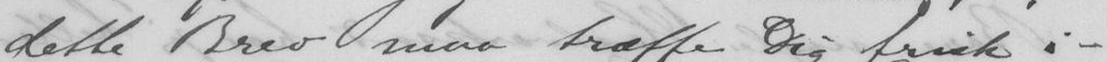dette Brev maa træffe Dig frisk i-
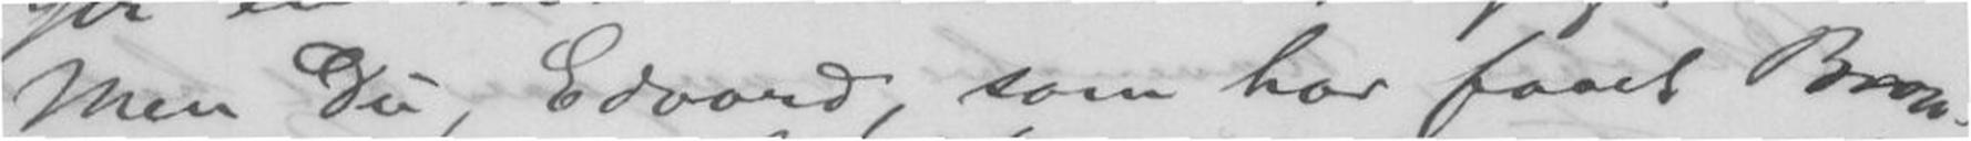Men Du, Edvard, som har faaet Bron-
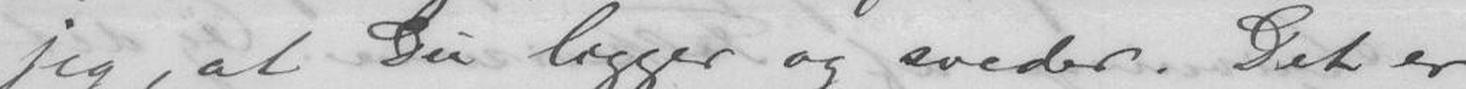jeg, at Du ligger og sveder. Det er
